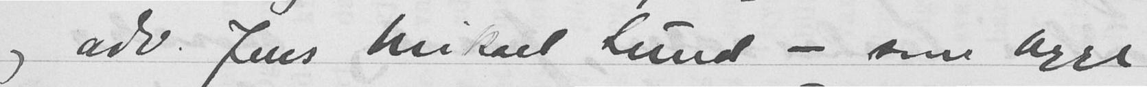og adv. Jens Mikkel Lund - som begge
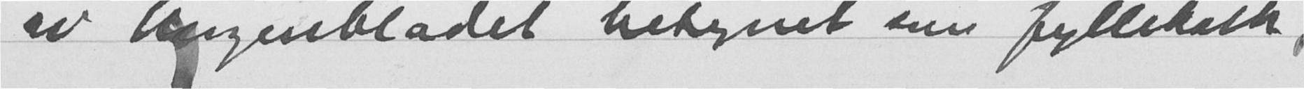av Morgenbladet bekynet som fyllekalk,
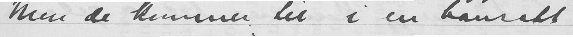Men de kommer til i en transitt
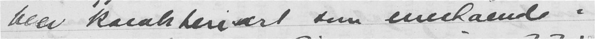blev karakterisert som enestående i
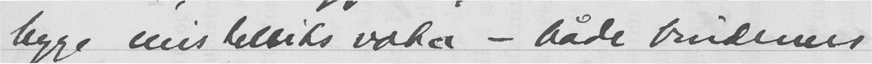begge mistillitsvota - både bøndernes
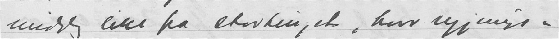middag like fra stortinget, hvor regjerings-
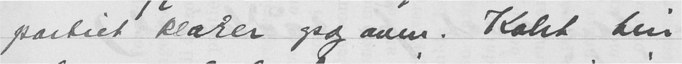partiet klarer opgaven. Koht blir
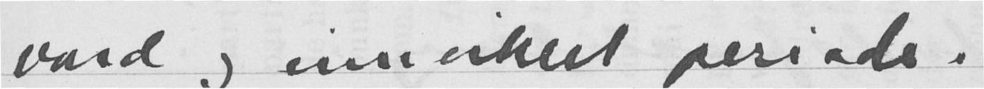vond og innviklet periode.
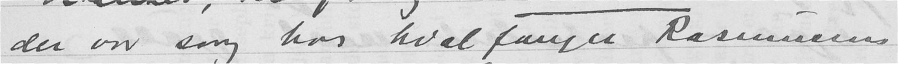der var sorg hos hvalfanger Rasmussen
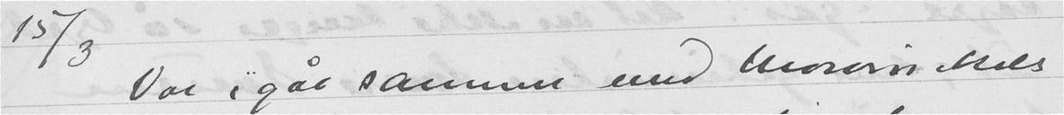15/3 Var igår sammen med Mowinckels
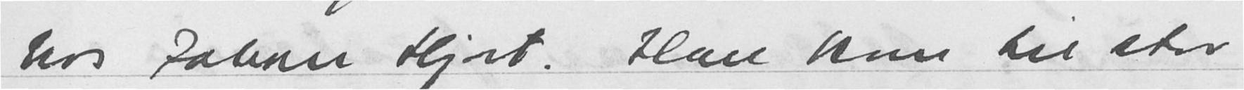hos Johan Hjort. Han kom til stor
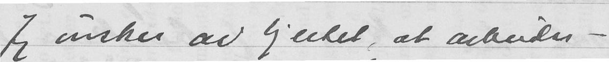Jeg ønsker av hjertet, at arbeider-
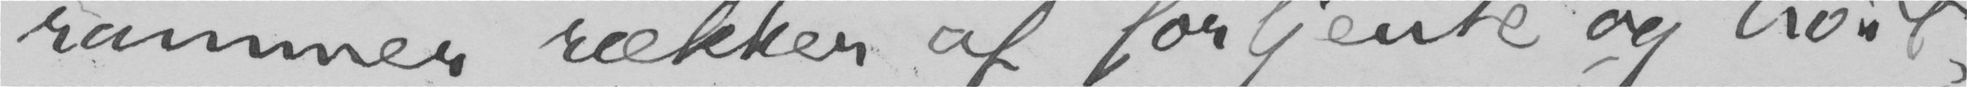rammer rækker af fortjente og høit-
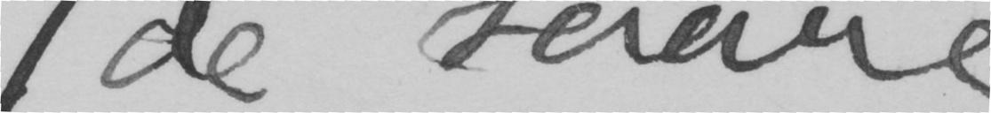de saare
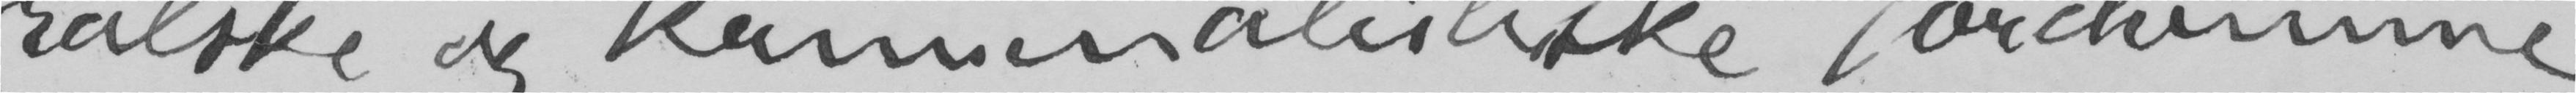ralske og kriminalistiske fordomme
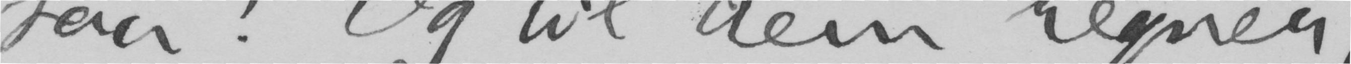saa! Og til dem regner
